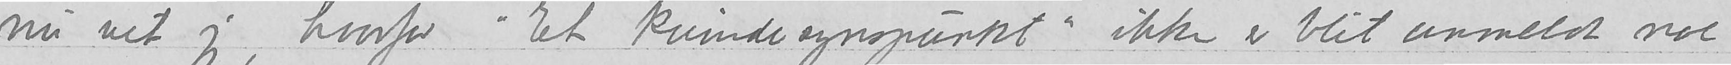nu vet jeg, hvorfor «Et kvindesynspunkt» ikke er blit anmeldt noe
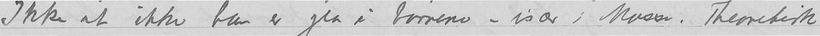Ikke at ikke han er glad i børnene – især i Mosse.  Theoretisk
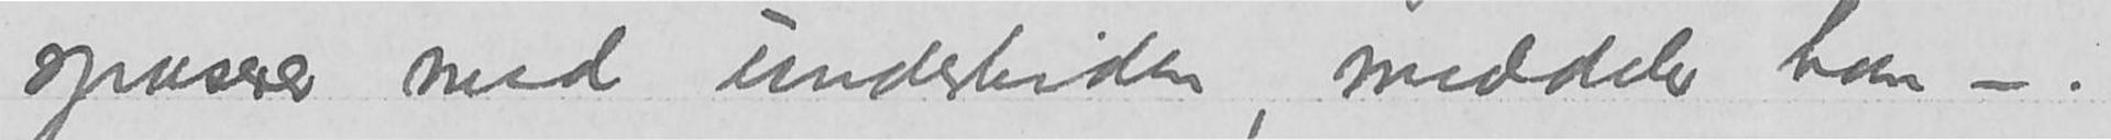spaserer med undertiden, meddeler han –.
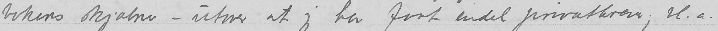bokens skjæbne – utover at jeg har faat en del privatbrever; bl.a.
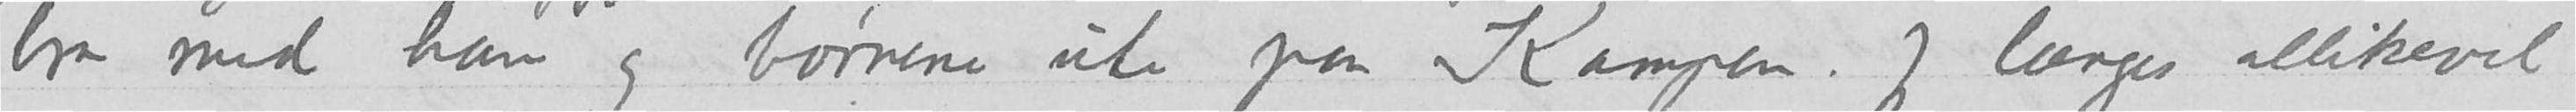bra med ham og børnene ute paa Kampen.  Jeg længes allikevel
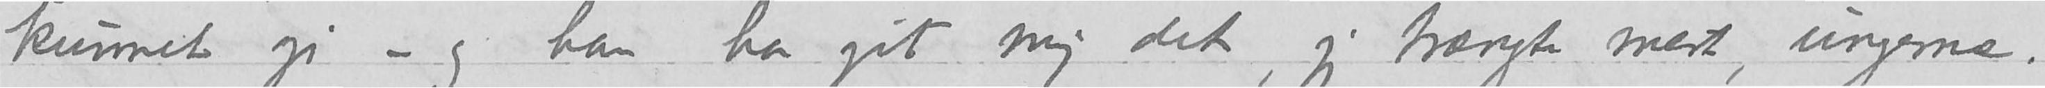kunnet gi – og han har git mig det, jeg trængte mest, ungerne.
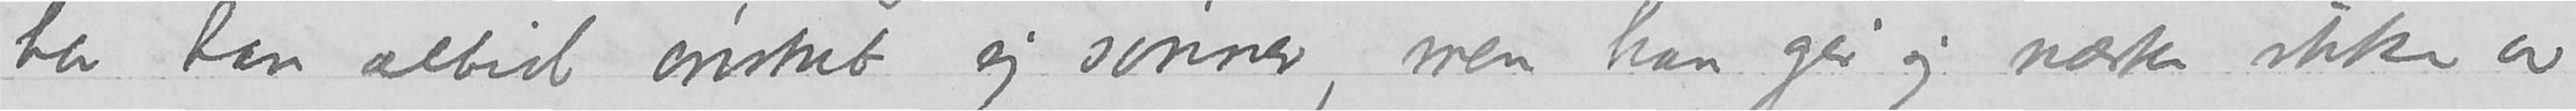har han altid ønsket sig sønner, men han gir seg næsten ikke av.
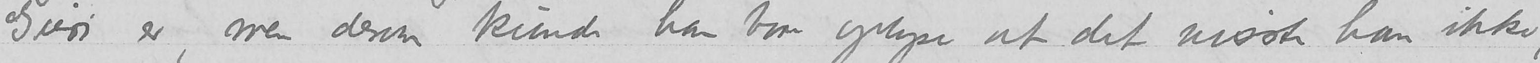Guri er, men derom kunde han bare oplyse at det visste han ikke,
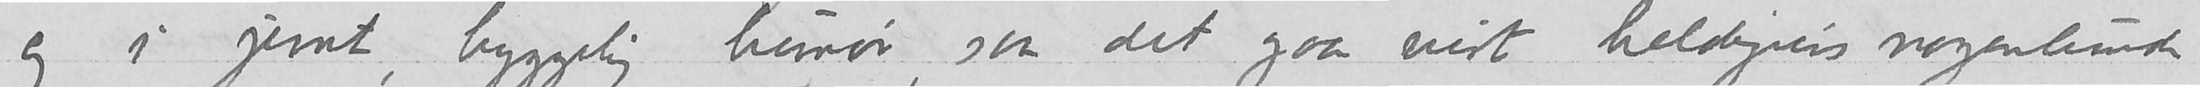og i jevnt, hyggelig humør, saa det gaar vist heldigvis nogenlunde
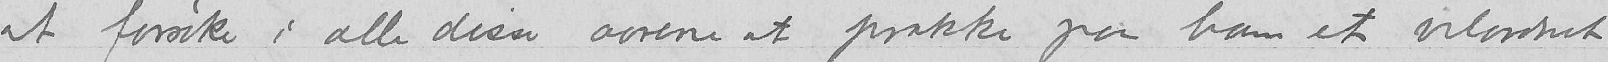at forsøke i alle disse aarene at prakke paa ham et velordnet
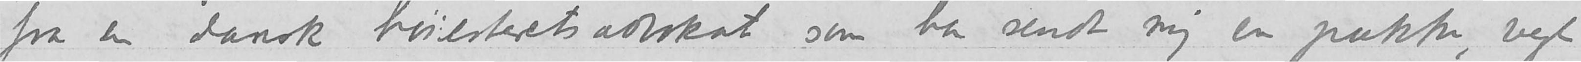ra en dansk høiesteretsadvokat som har sendt mig en pakke, vegt
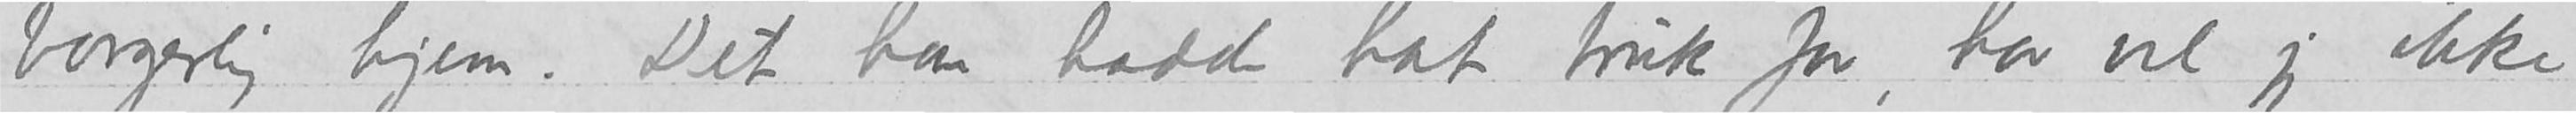borgerlig hjem. Det han hadde hat bruk for, har vel jeg ikke
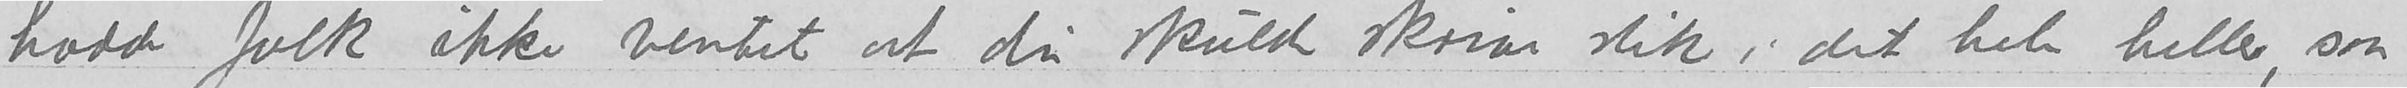hadde folk ikke ventet at du skulde skrive slik i det hele heller, saa
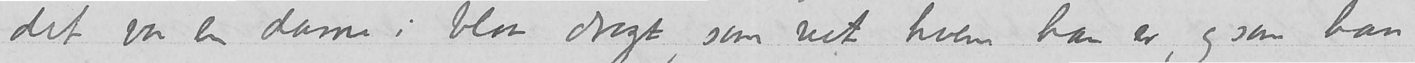det var en dame i blaa dragt, som vet hvem han er, og som han-
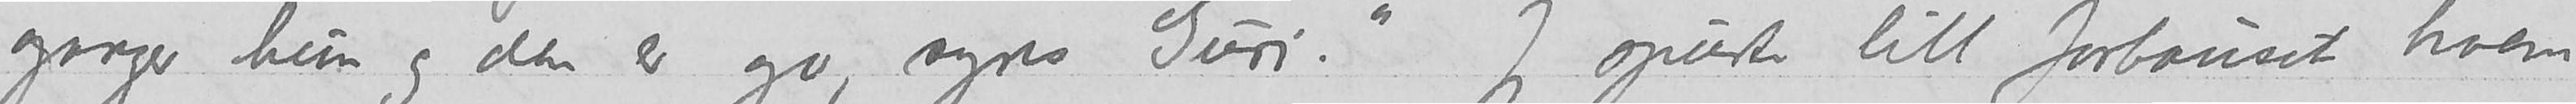ganger hun og den er go, syns Guri.»  Jeg spurte litt forbauset hvem
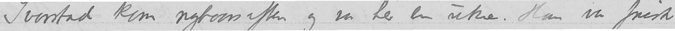Svarstad kom nytaarsaften og var her en uke. Han var frisk
